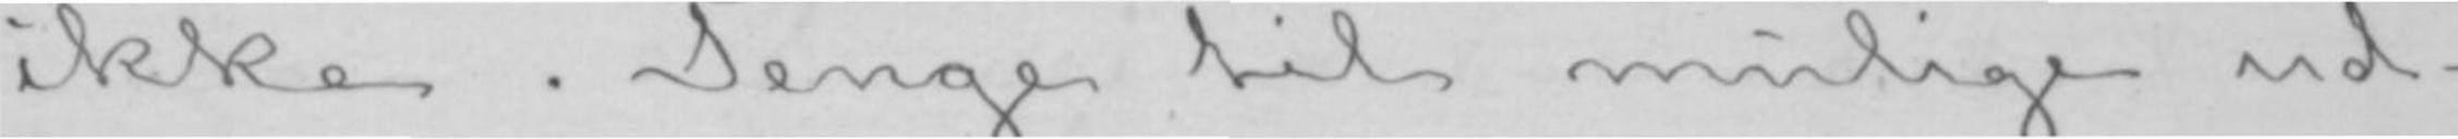ikke. Penge til mulige ud-
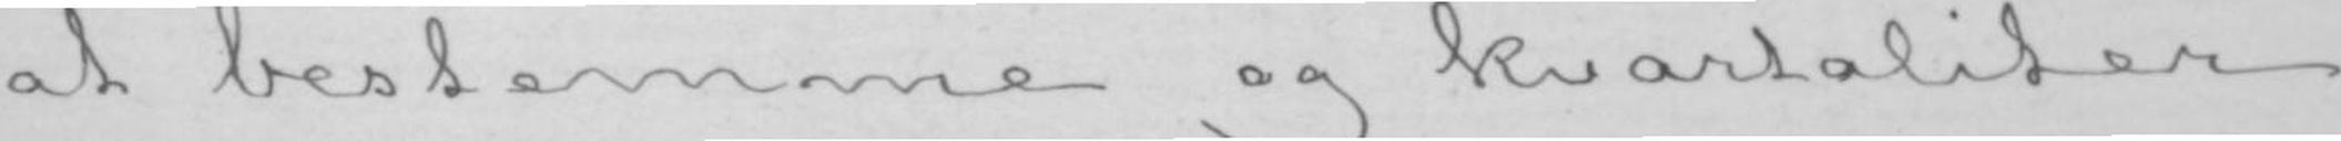at bestemme og kvartaliter
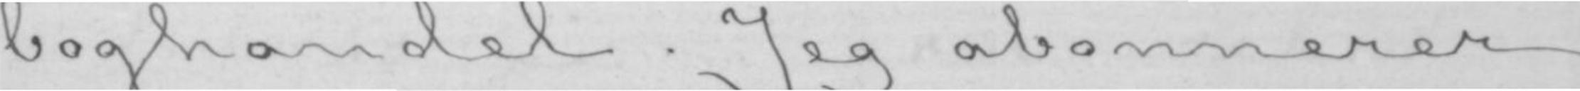boghandel. Jeg abonnerer
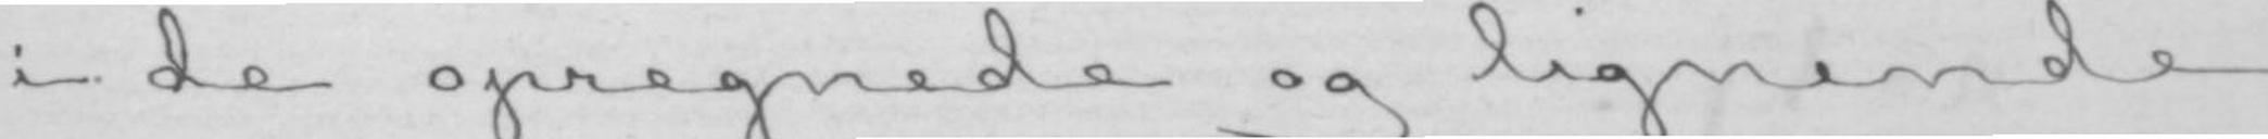i de opregnede og lignende
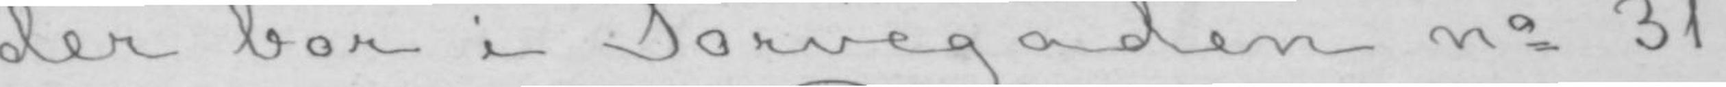der bor i Torvegaden no 31,
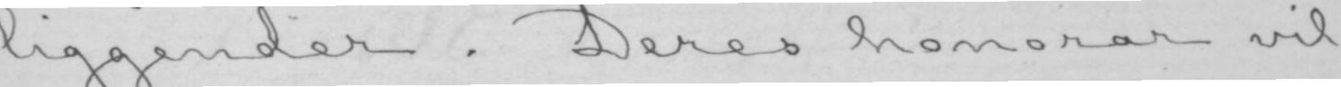liggender. Deres honorar vil-
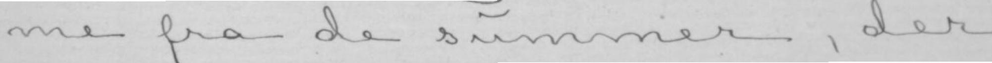me fra de summer, der
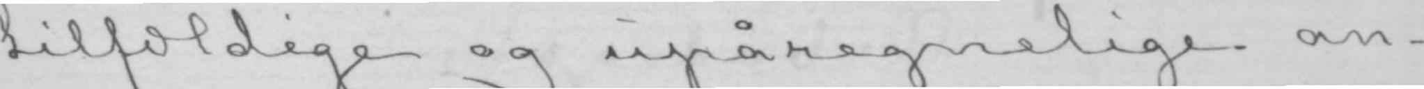tilfældige og upåregnelige an-
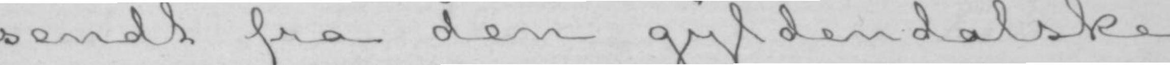sendt fra den gyldendalske
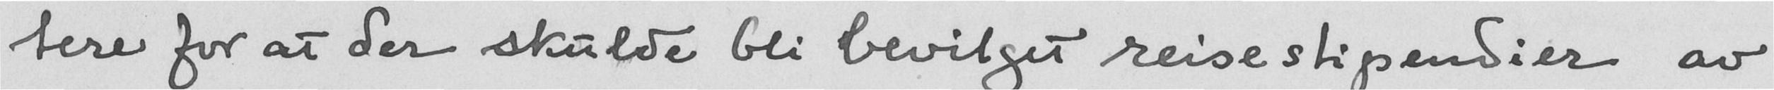tere for at der skulde bli bevilget reisestipendier av
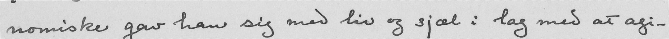nomiske gav han sig med liv og sjæl i lag med at agi-
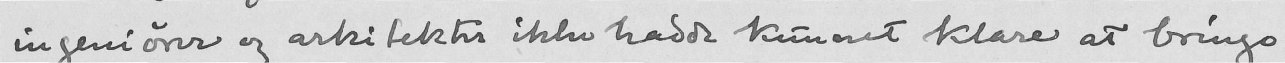ingeniører og arkitekter ikke hadde kunnet klare at bringe
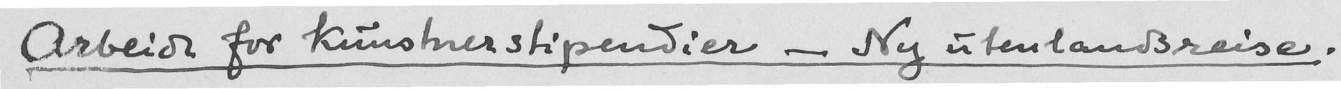Arbeide for kunstnerstipendier - Ny utenlandsreise.
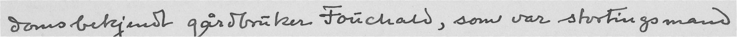doms bekjendt gårdbruker Fouchald, som var stortingsmand
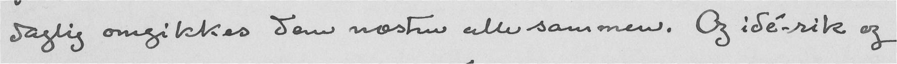daglig omgikkes dem næsten alle sammen. Og idé-rik og
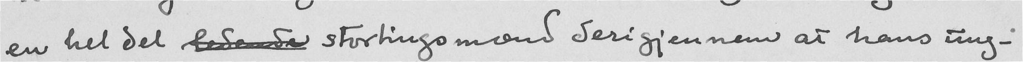en hel del ledende stortingsmænd derigjennem at hans ung-
